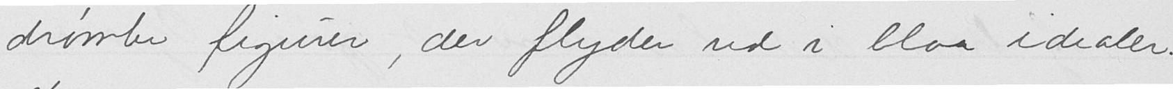drømte figurer, der flyder ud i blaa idealer.
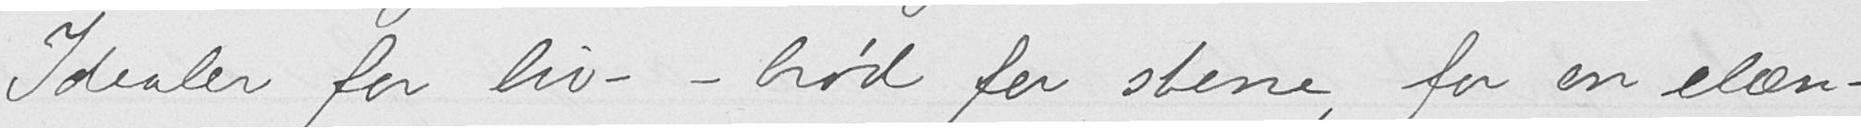Idealer for liv - brød for stene, for en elæn-
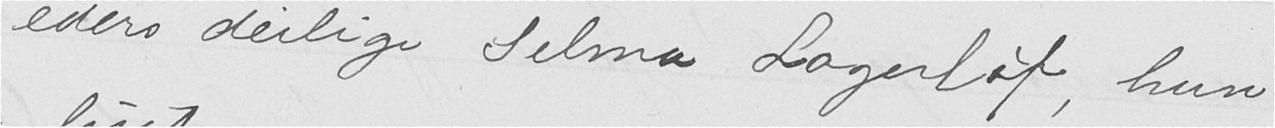- eders deilige Selma Lagerløf, hun
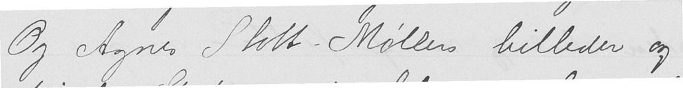Og Agnes Slott-Møllers billeder og
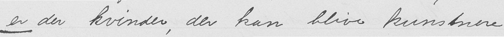er der kvinder, der kan blive kunstnere
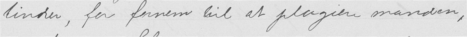tindrer, for fornem til at plagiere manden,
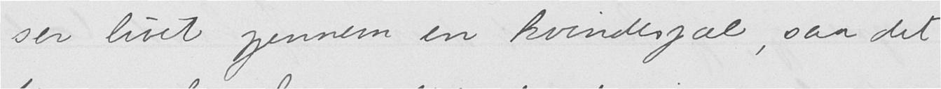ser livet gjennem en kvindesjæl, saa det
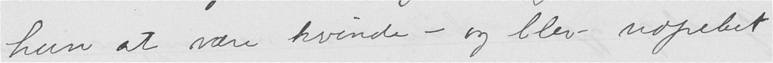hun at være kvinde - og ble udpebet
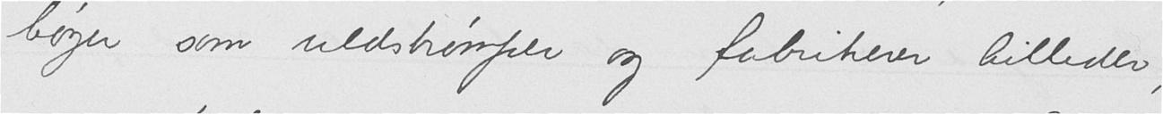bøger som uldstrømper og fabrikerer billeder,
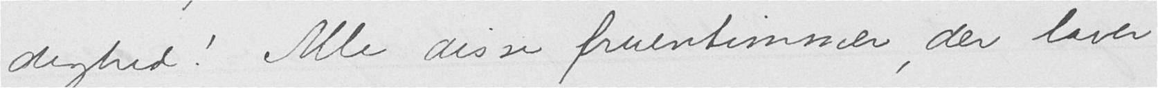dighed! Alle disse fruentimmer, der laver
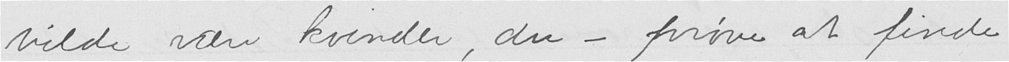vilde være kvinder, du - prøve at finde
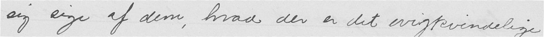sig sige af dem, hvad der er det evigkvindelige
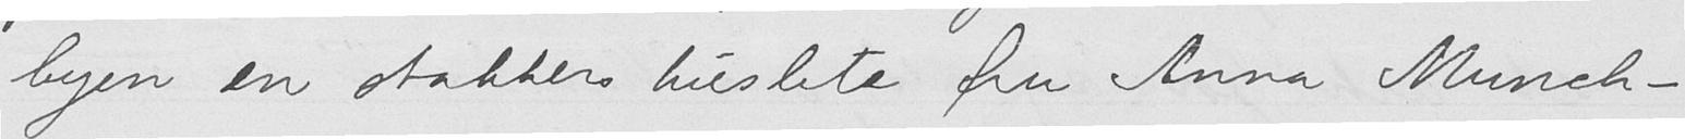byen en stakkars tuslete fru Anna Munch -
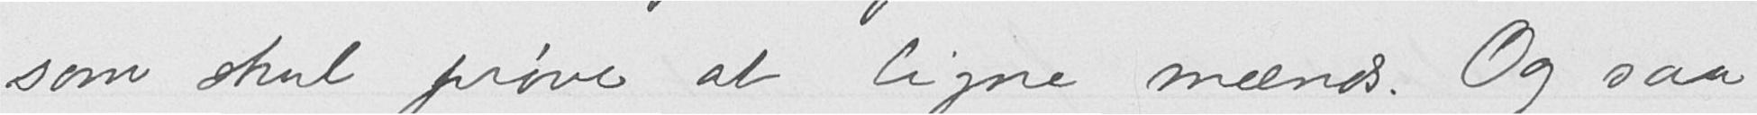som skal prøve at ligne mænds. Og saa
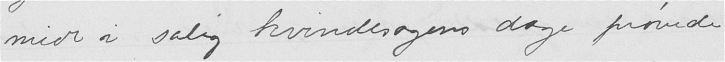midt i salig kvindesagens dage prøvede
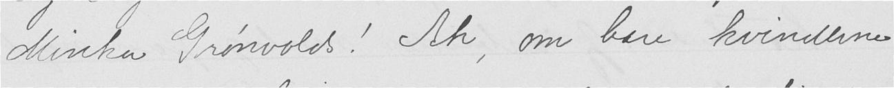Minka Grønvolds! Ak, om bare kvinderne
